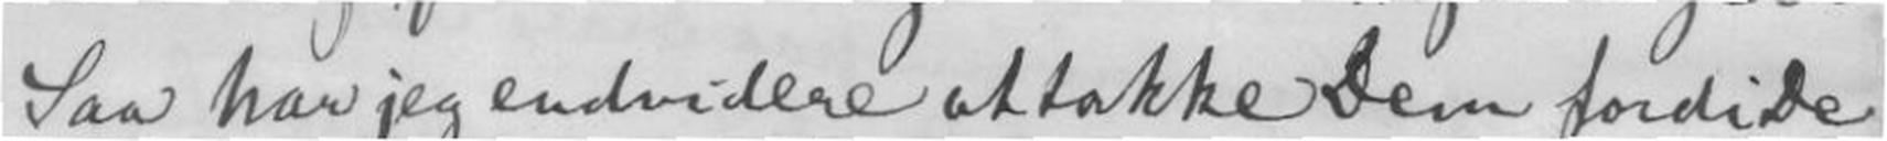Saa har jeg endvidere at takke Dem fordi De
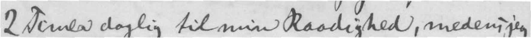2 Timer daglig til min Raadighed, medem jeg
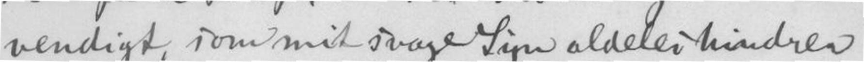vendigt, som mit svage Syn aldeles hindrer
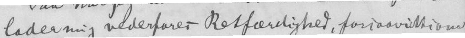lader mig vederfares Retfærdighed, forsaavidt som
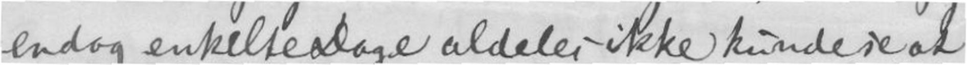endog enkelte Dage aldeles ikke kunde se at
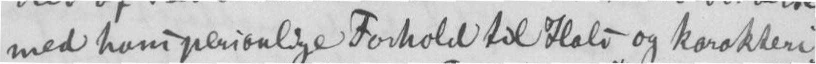med hans personlige Forhold til Hals og Karateri-
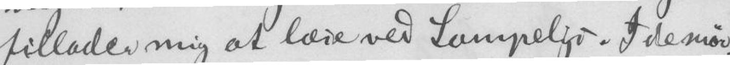tillader mig at læse ved Lampelys. I de mør-
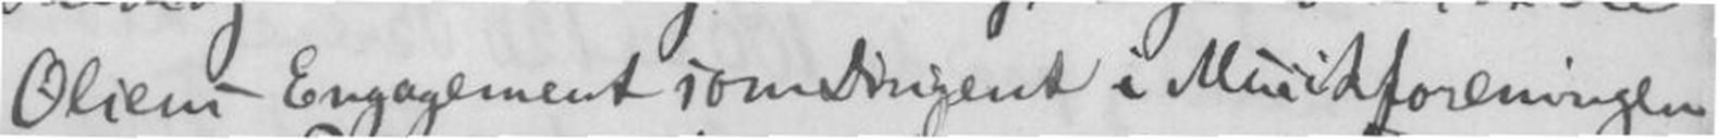Olsen Engagement som Dirigent i Musikforeningen
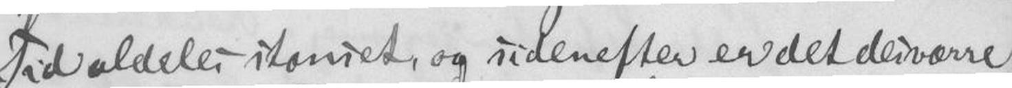Tid aldeles stanset, og siden efter er det desværre
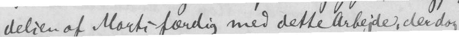delsen af Marts Færdig med dette Arbejde, der dog
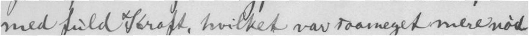med fuldt Kraft, hvilket var saameget mere nød-
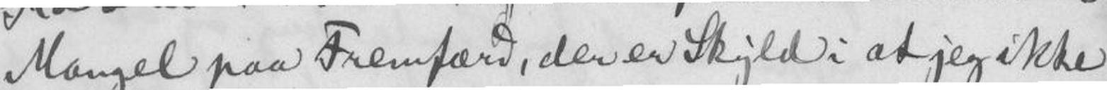Mangel paa Fremfærd, der er Skyld i at jeg ikke
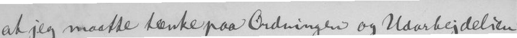at jeg maatte tænke paa Ordningen og Udarbejdelsen
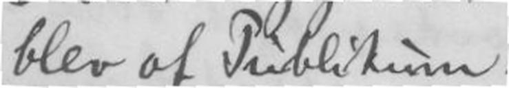blev af Publikum
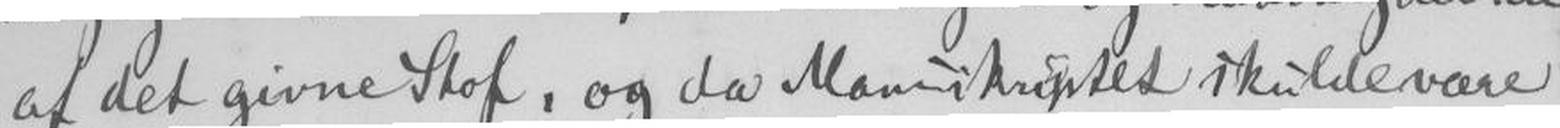af det givne Stof, og da Manuskriptet skulde være
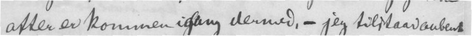atter er kommen igang derned, - jeg tilstaar aabent
